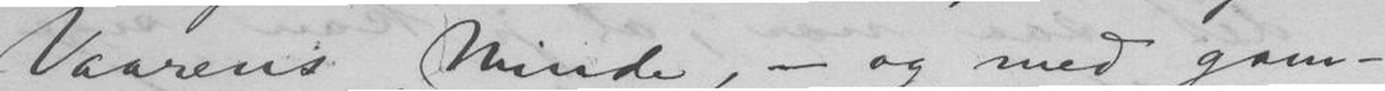Vaarens Minde, - og med gam-
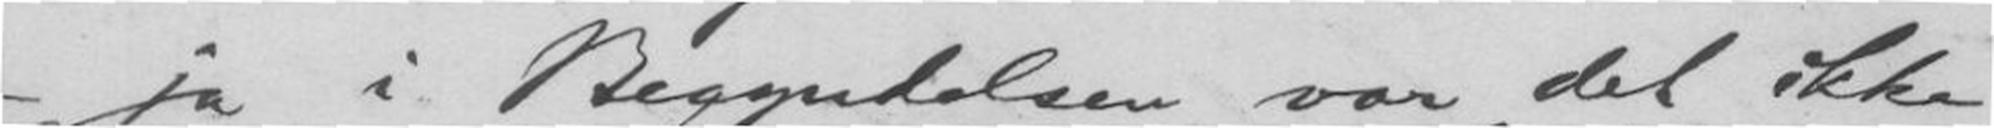- ja i Begyndelsen var det ikke
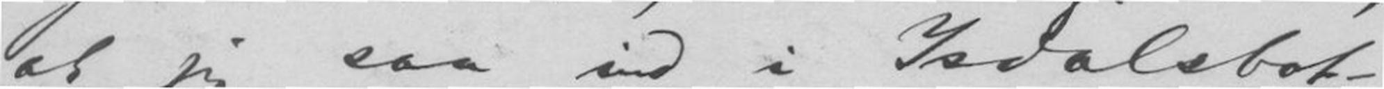at jeg saa ind i Isdalsbot-
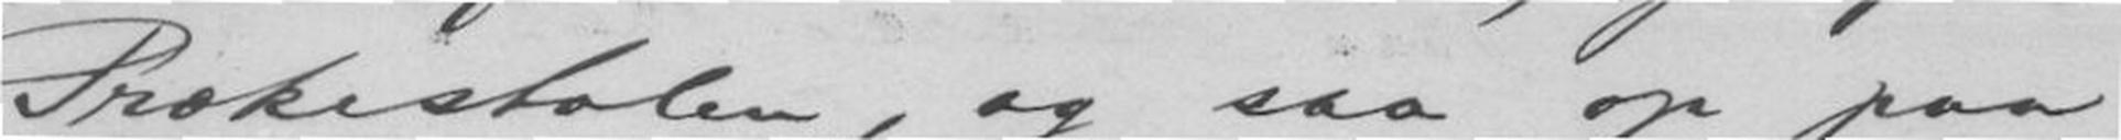Prækestolen, og saa op paa
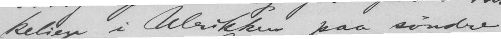kelien i Ulrikken paa søndre
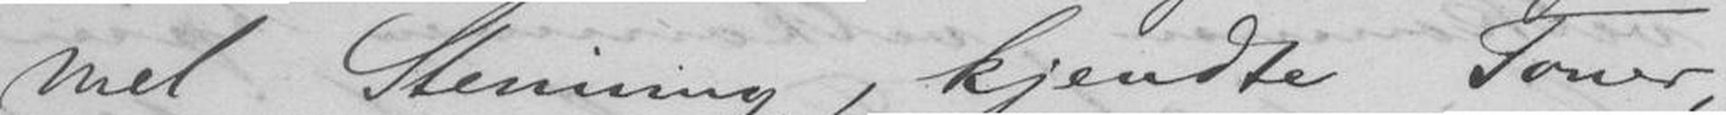mel Stemning, kjendte Toner,
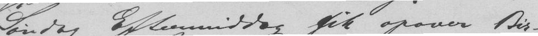Søndag Eftermiddag gik opover Bir-
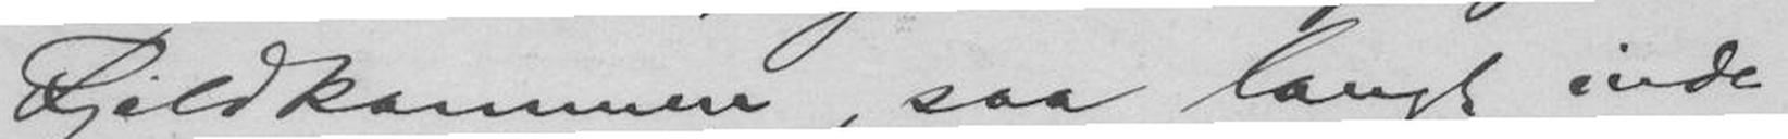Fjeldkammen, saa langt inde,
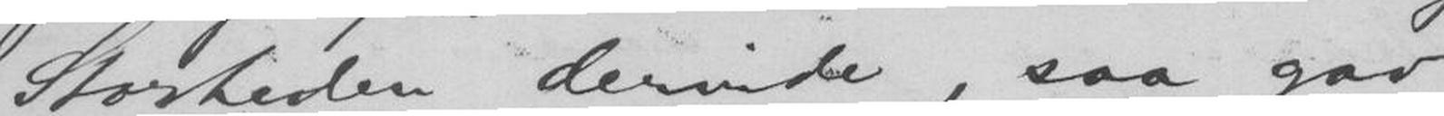Storheden derinde, saa gav
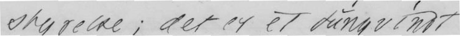styrelse; det er et tungvindt
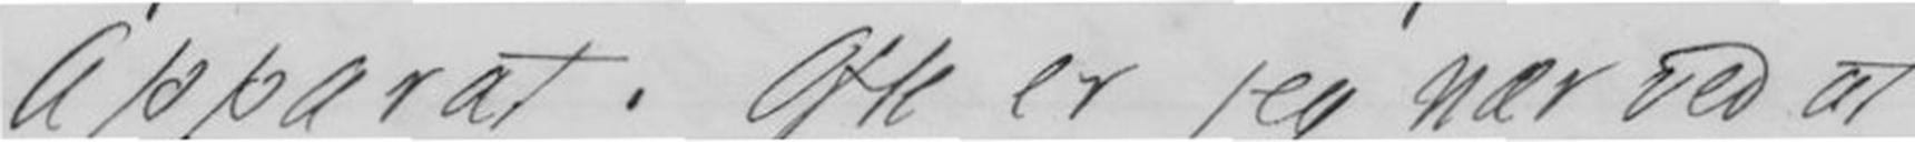Apparat. Ofte er jeg nær ved at
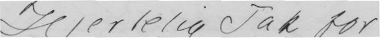Hjertelig Tak for
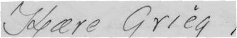Kjære Grieg,
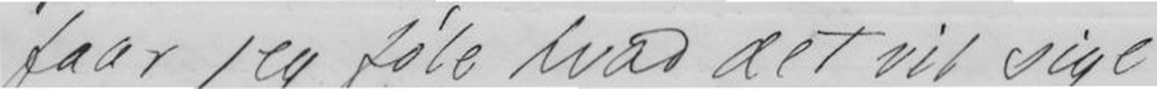faar jeg føle hvad det vil sige
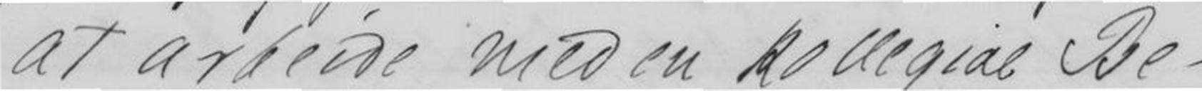at arbeide med en kollegial Be-
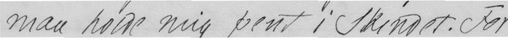maa holde mig pent i skindet. For
